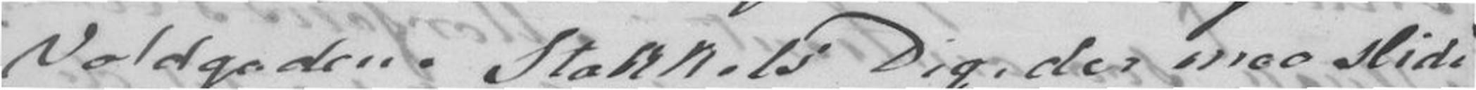Voldgaden. Stakkels Dig, der maa slide
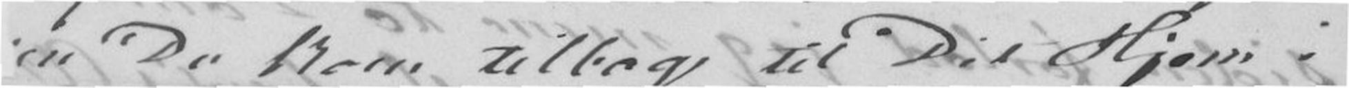den Du kom tilbage til Dit Hjem i
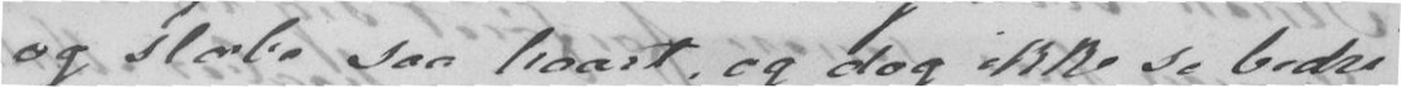og slæbe saa haart, og dog ikke se bedre
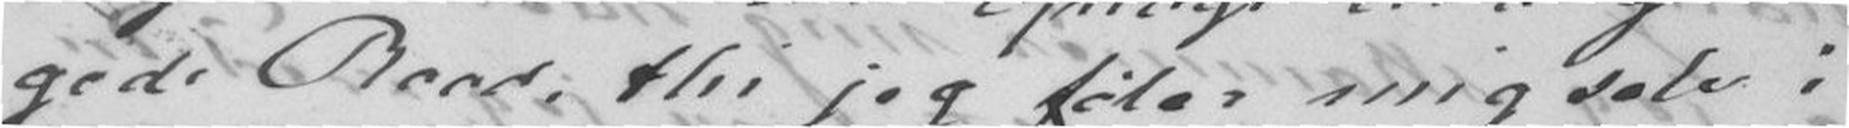gode Raad, thi jeg føler mig selv i
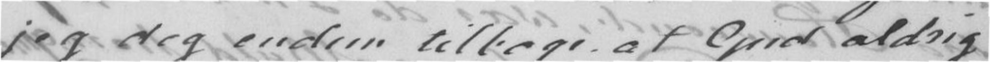jeg dog endnu tilbage at Gud aldrig
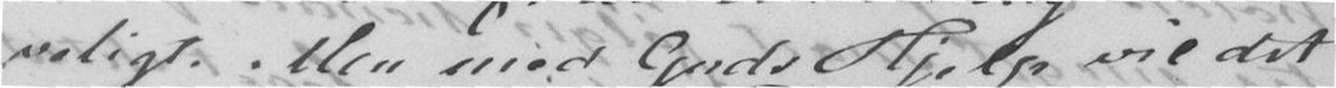veligt. Men mod Guds Hjelp vil det
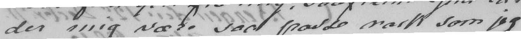der mig være saa passe rask som jeg
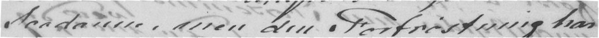saadanne, men den Fortrøstning har
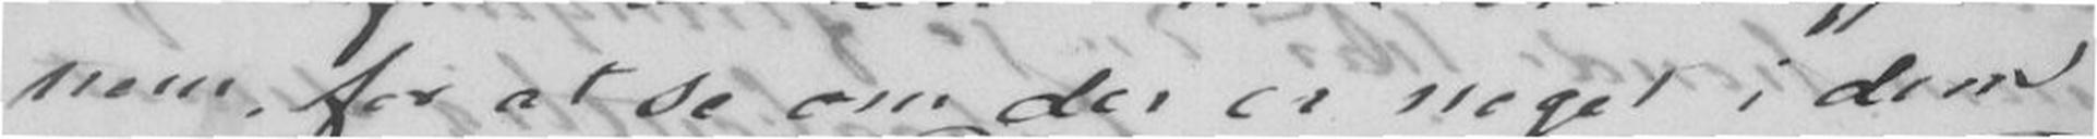nem for at se om der er noget i dem
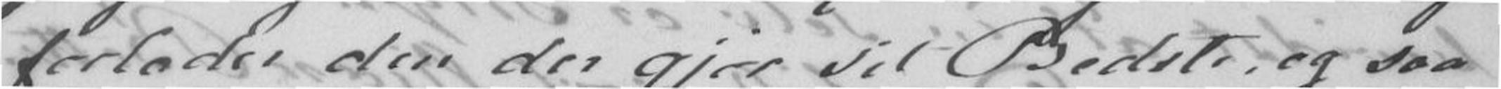forlader den der gjør sit Bedste, og saa
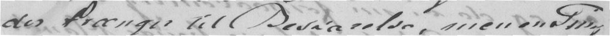der trænger til Besvarelse, men en Ting
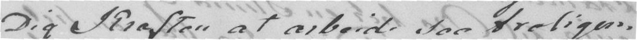Dig Kraften at arbeide saa troligen,
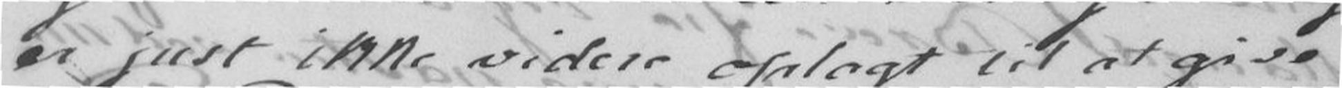er just ikke videre oplagt til at give
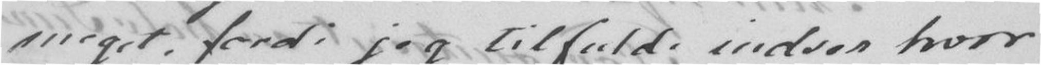meget, fordi jeg tilfulde indser hvor
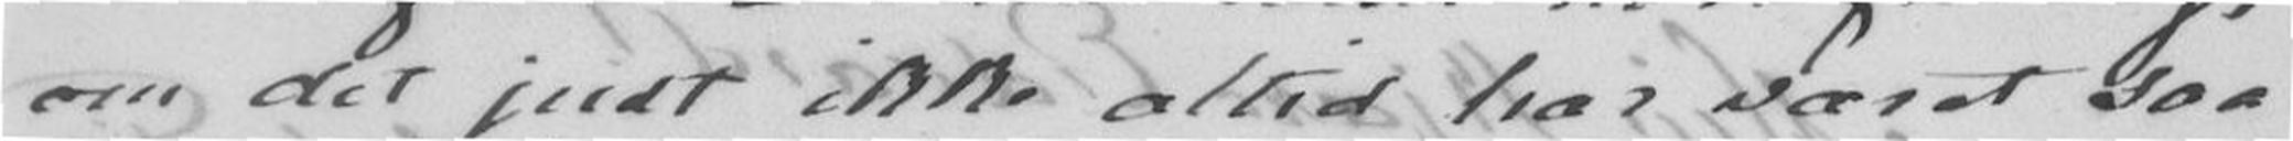om det just ikke altid har været saa
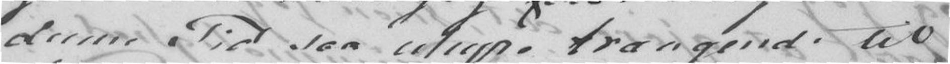denne Tid saa uhyre trængende til
